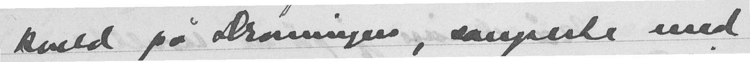kveld på Dronningen, sanpeste med
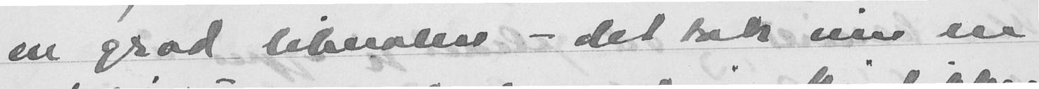en grad liberalere - det tok inn en
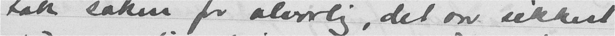tar saken for alvorlig, det var sikkert
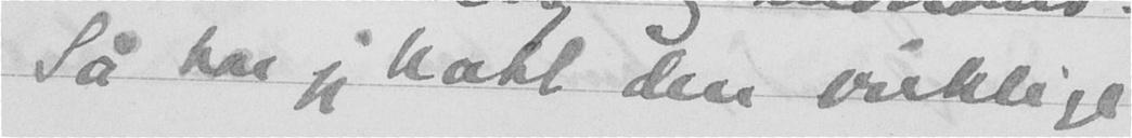Så har jeg hatt den virklige
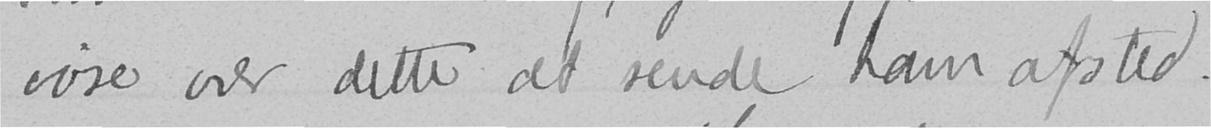vøse over dette at sende ham afsted.
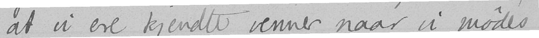at vi ere kjendte venner, naar vi mødes.
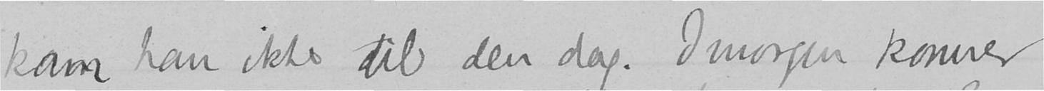kom han ikke til den dag. Imorgen kommer
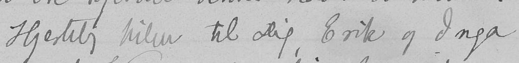Hjertelig hilsen til Dig, Erik og Inga
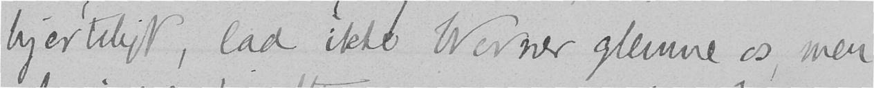hjerteligt, lad ikke Werner glemme os, men
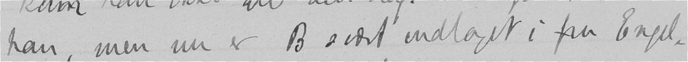han, men nu er B svært indtaget i fru Engel-
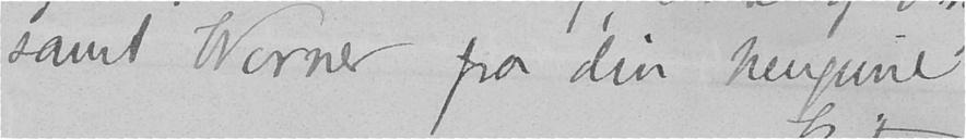samt Werner fra din hengivne
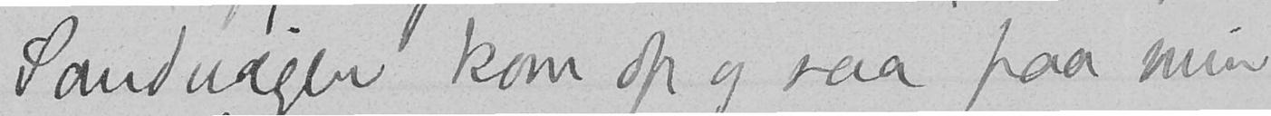Sandvigen kom op og saa paa min
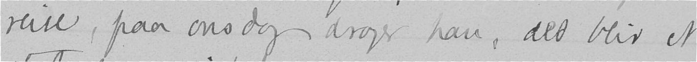reise, paa onsdag drager han, det blir et
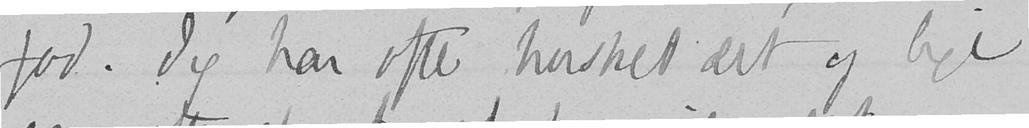fod. Jeg har ofte husket det og lige
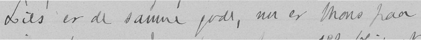Lies er de samme gode, nu er Mons paa
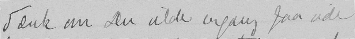Tænk om Du vilde engang faa vide
